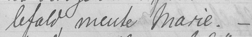lefald mente Marie. -
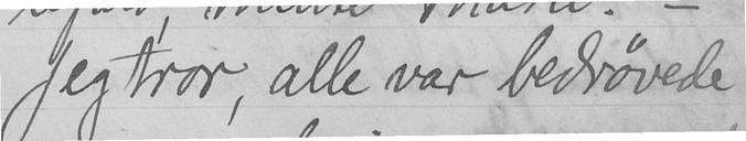Jeg tror, alle var bedrøvede
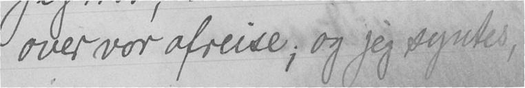over vor afreise; og jeg syntes,
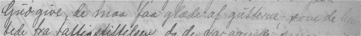Gud give, de maa faa glæde af gutterne, som de hen-
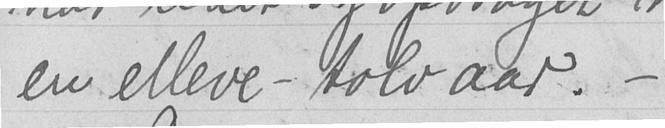en elleve- tolv aar. -
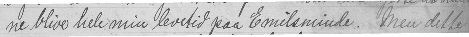ne blive hele min levetid paa Emilsminde. Men dette
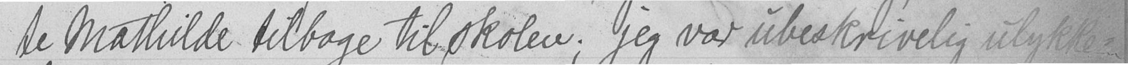te Mathilde tilbage til skolen; jeg var ubeskrivelig ulykke-
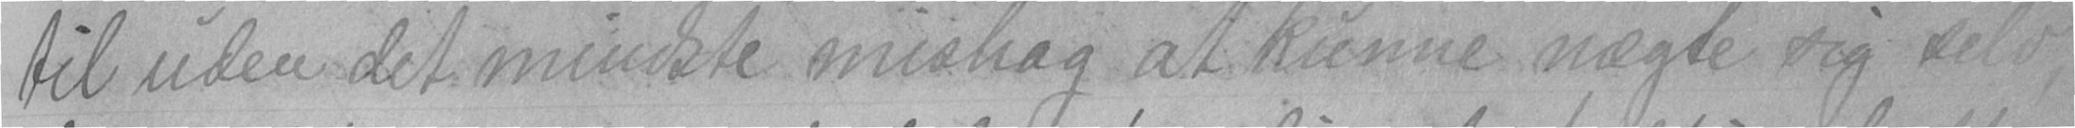til uden det mindste mishag at kunne nægte sig selv,
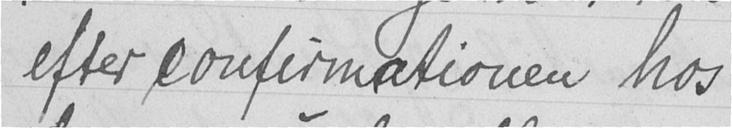efter confirmationen hos
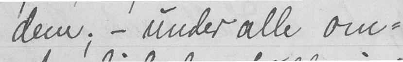dem; - under alle om-
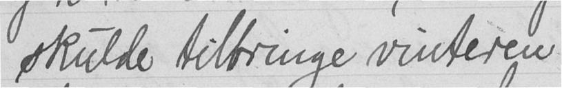skulde tilbringe vinteren
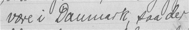være i Danmark, saa der
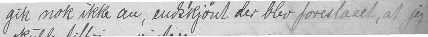gik nok ikke an, endskjønt der blev foreslaaet, at jeg
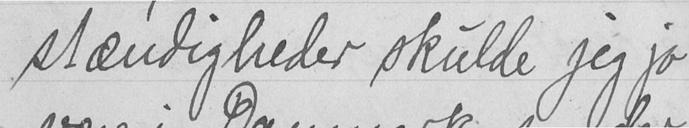stændigheder skulde jeg jo
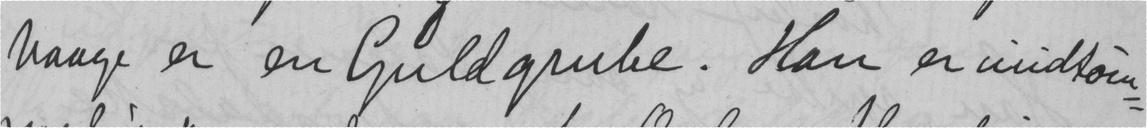vaage er en Guldgrube. Han er uudtøm-
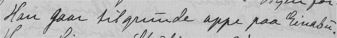Han gaar tilgrunde oppe paa Einabu.
